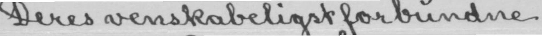Deres venskabeligst forbundne
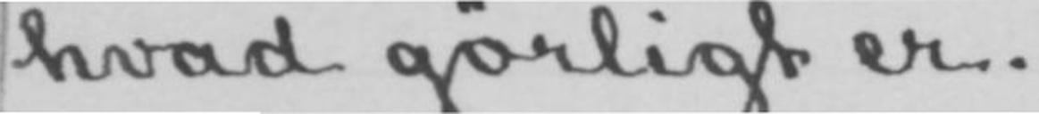hvad gørligt er.
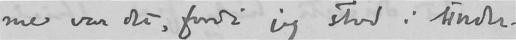me var det, fordi jeg stod i under-
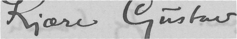Kjære Gustav
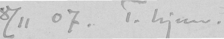8/11 07. T.hjem.
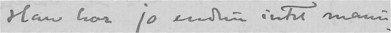Han har jo endnu intet manu-
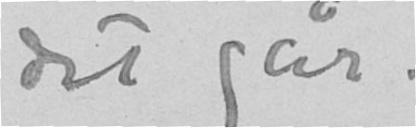det går.
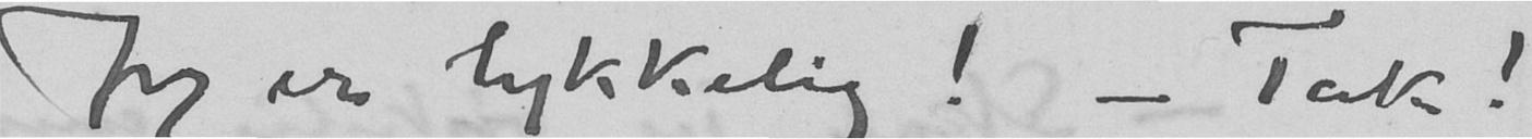Jeg er lykkelig! - Tak!
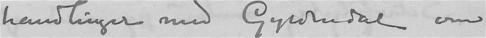handlinger med Gyldendal om
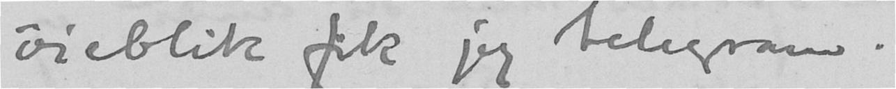øieblik fik jeg telegram.
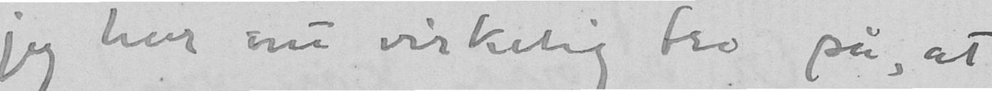jeg har nu virkelig tro på, at
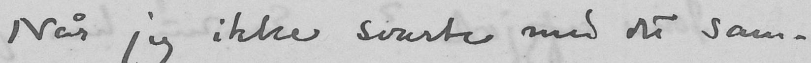Når jeg ikke svarte med det sam-
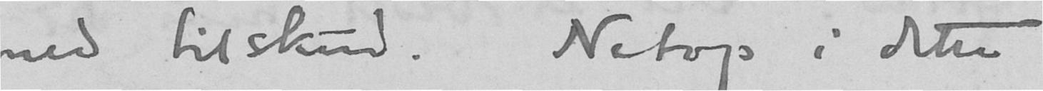med tilskud. Netop i dette
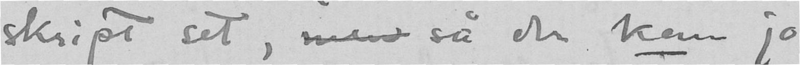skript set, men så der kan jo
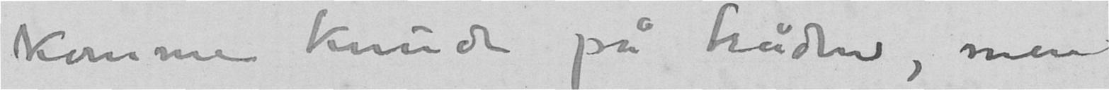komme knude på tråden, men
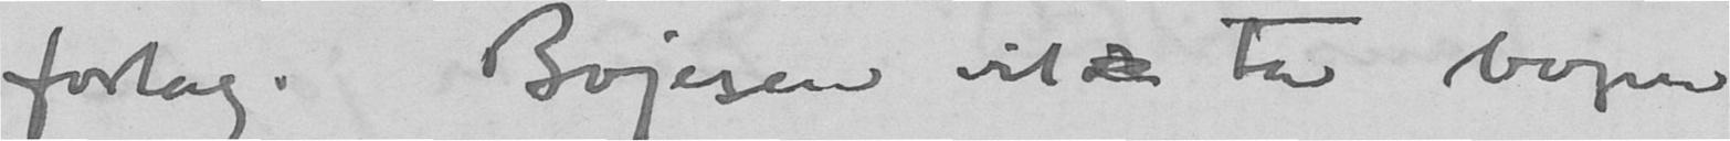forlag. Bojesen vilde ta bogen
